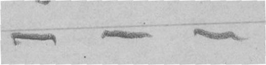- - -
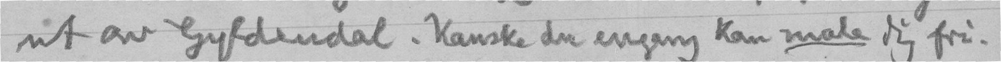ut av Gyldendal. Kanske du engang kan male dig fri.
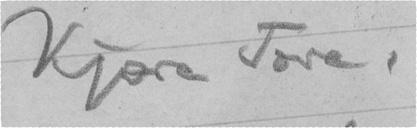Kjære Tore,
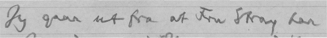Jeg gaar ut fra at Fru Stray har
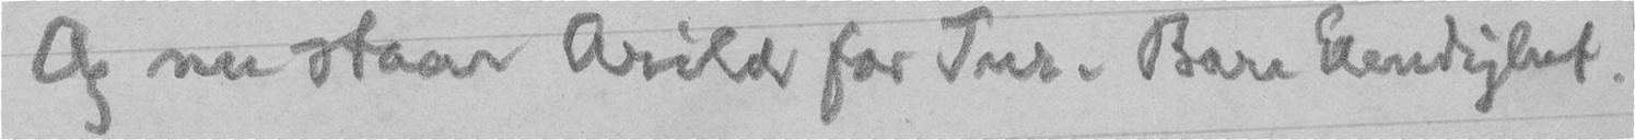Og nu staar Arild for Tur. Bare Elendighet.
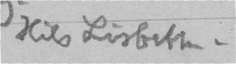Hils Lisbeth.
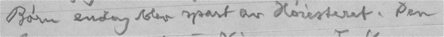Børn endog blev spart av Høiesteret. Den
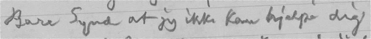Bare Synd at jeg ikke kan hjelpe dig
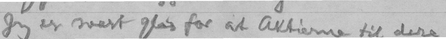Jeg er svært glad for at Aktierne til dere
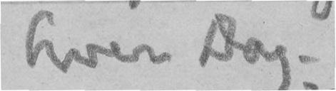hver Dag.
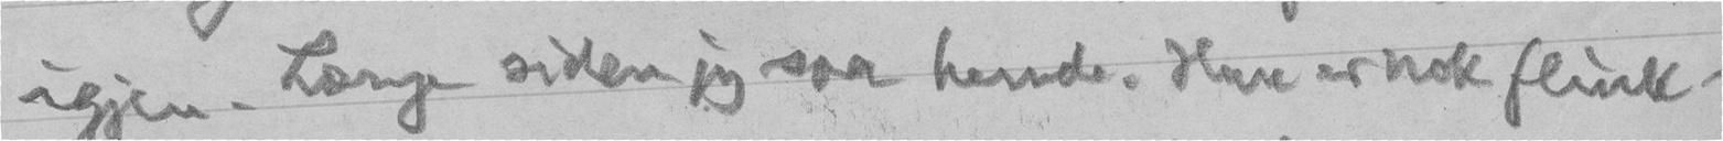igjen. Længe siden jeg saa hende. Hun er nok flink.
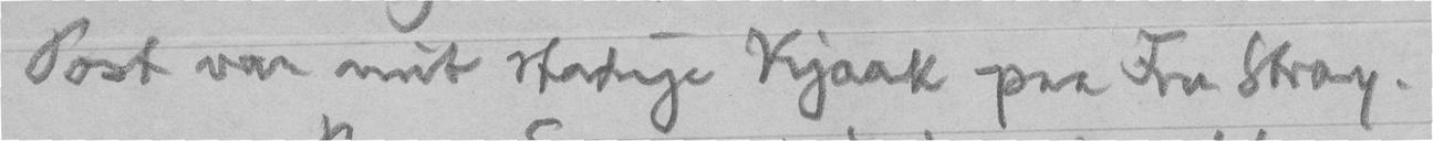Post var mit stadige Kjaak paa Fru Stray.
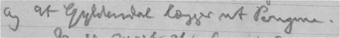og at Gyldendal lægger ut Pengene.
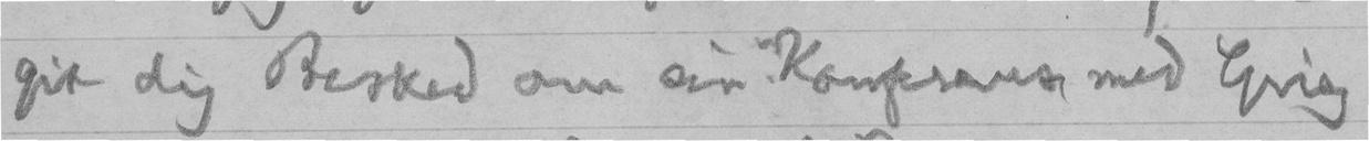git dig Besked om sin Konferanse med Grieg
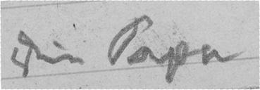Din Papa
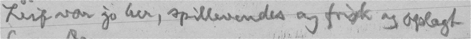Leif var jo her, spillevendes og frisk og oplagt
